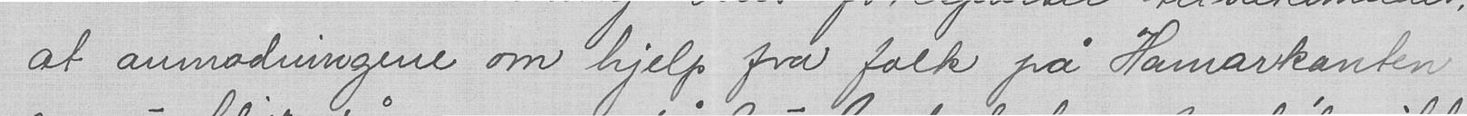at anmodningene om hjelp fra folk på Hamarkanten
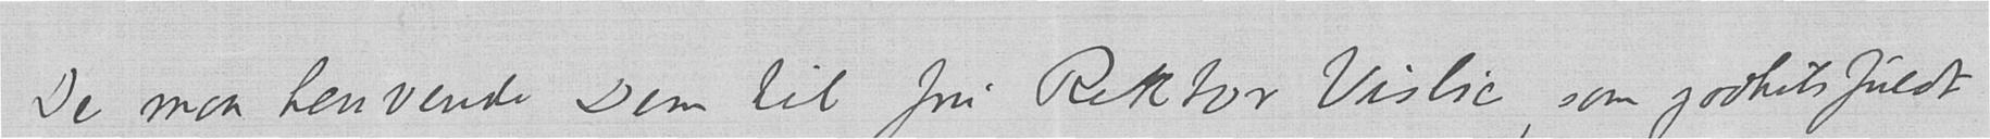De maa henvende Dem til fru Rektor Vislie som godhetsfuldt
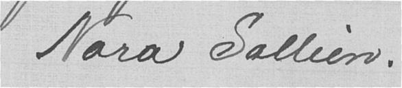Nora Sallien.
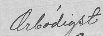Ærbødigst
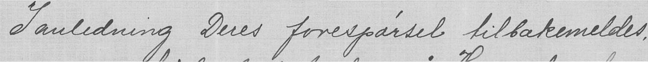I anledning Deres forespørsel tilbakemeldes,
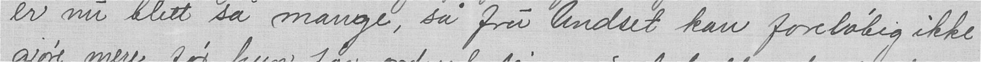er nu blitt så mange, så fru Undset kan foreløbig ikke
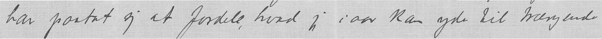har paatat sig at fordele, hvad jeg i aar kan yde til trængende
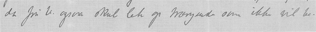da fru V. ogsaa skal lete op trængende som ikke vil be.
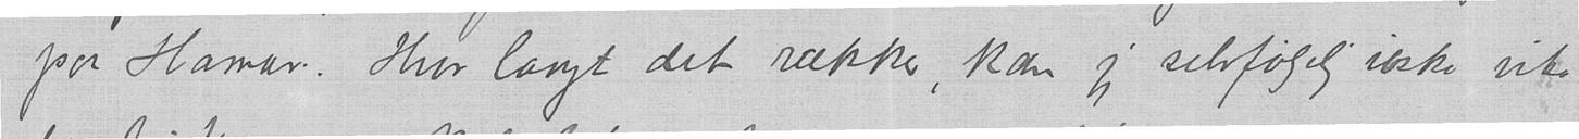paa Hamar. Hvor langt det rækker kan jeg selvfølgelig ikke vite
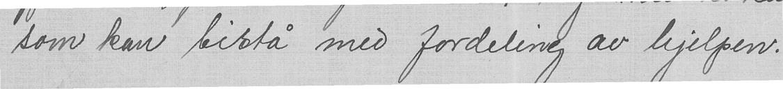som kan bistå med fordeling av hjelpen.
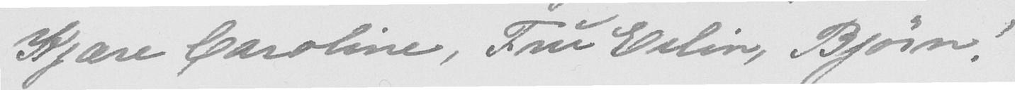Kjære Caroline, Fru Eclin, Bjørn!
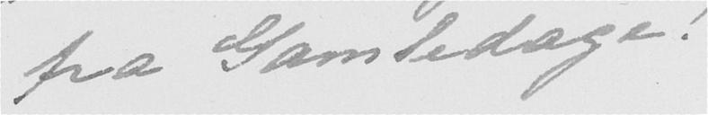fra Gamledage!
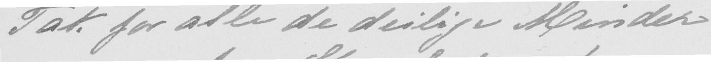Tak for alle de deilige Minder
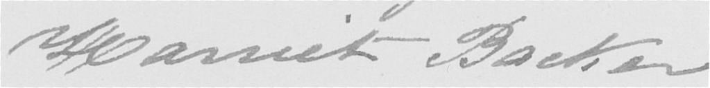Harriet Backer
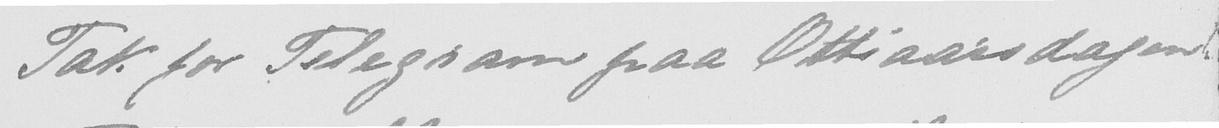Tak for Telegram paa Ottiaarsdagen!
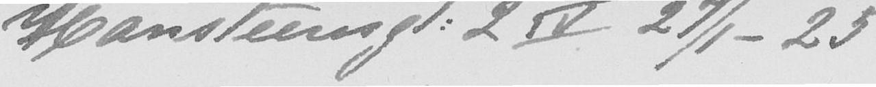Hansteensgt. 2 IV 27/1 - 25
